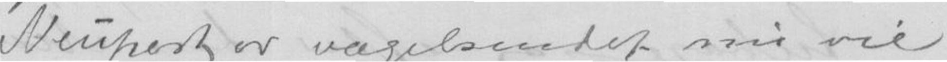Neupert er vægelsindet nu vil
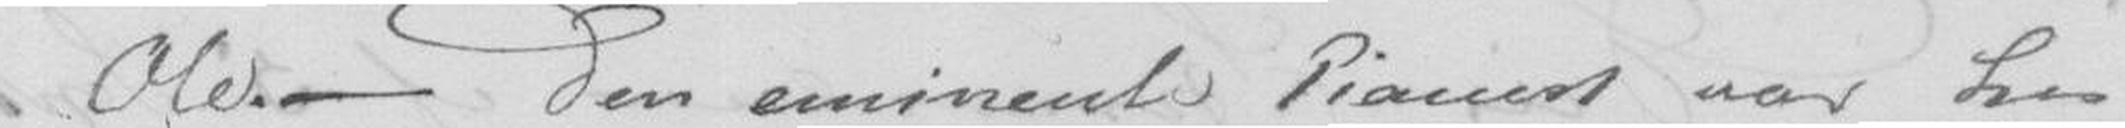Ole. - Den eminente Pianist var hos
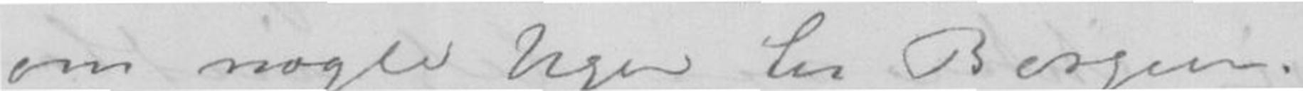om nogle Uger til Bergen.
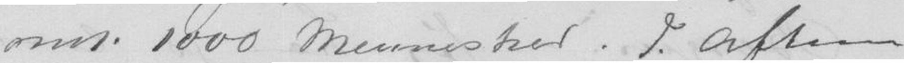omt. 1000 Mennesker. I Aften
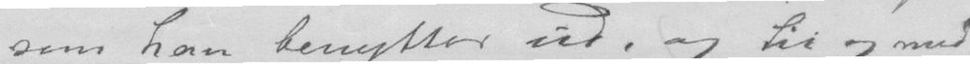som han benytter ud, og til og med
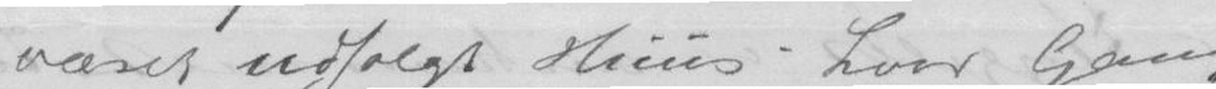været udsolgt Huus hver Gang
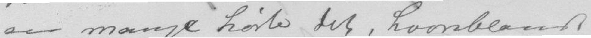saa mange hørte det, hvoriblandt
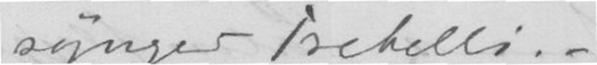synger Trebells. -
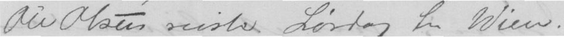Ole Olsen reiste Lørdag til Wien.
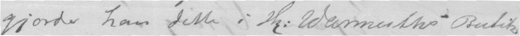gjorde han dette i Hr: Warmuths Butik
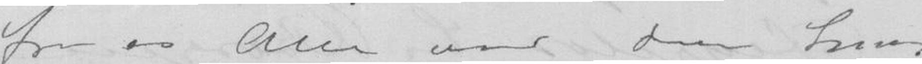fra os alle ved Din heng
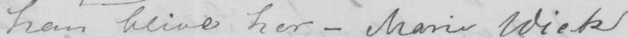han blive her - Marie Wick
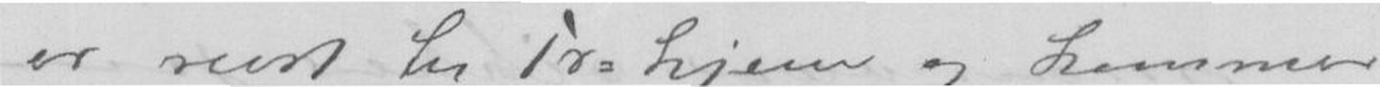er reist til Tr=hjem og kommer
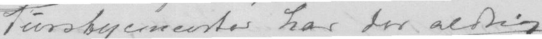Tursbyconcerter har der aldrig
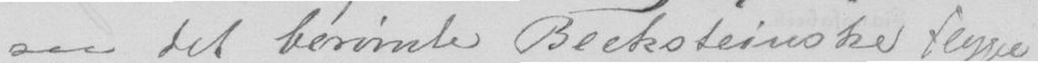saa det berømte Becksteinske Flygel
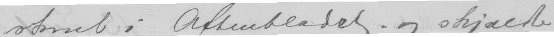skrut i Aftenbladet og skjældte
